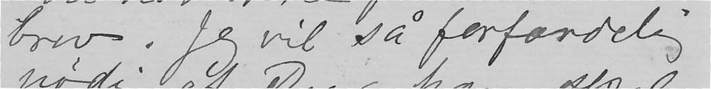brev. Jeg vil så forfærdelig
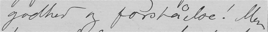godhed og forståelse! Men
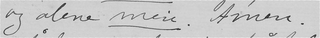og alene min. Amen.
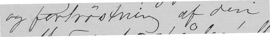og fortrøstning af din
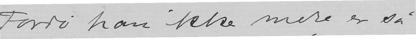Fordi han ikke mere er så
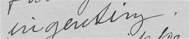ingenting.
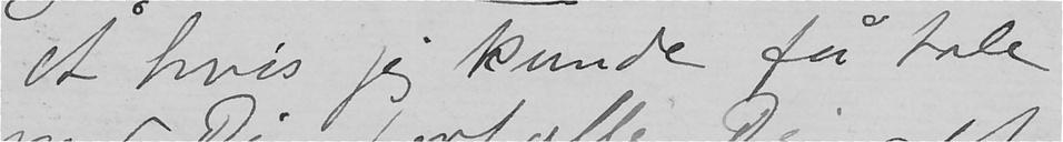Å hvis jeg kunde få tale
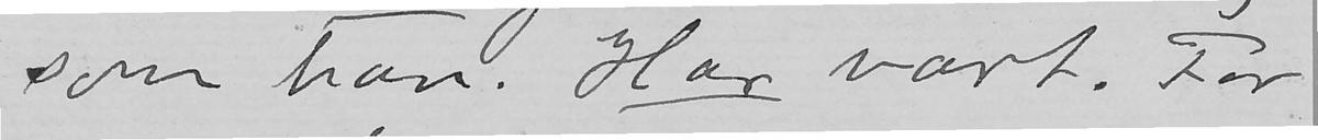som han. Har vært. For
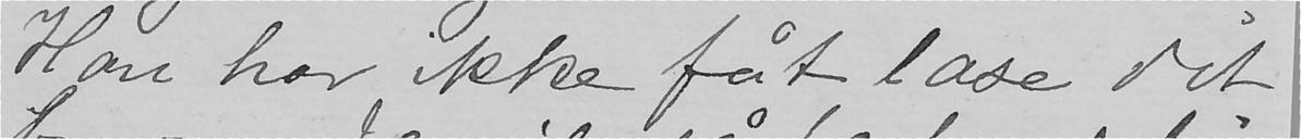Han har ikke fåt læse dit
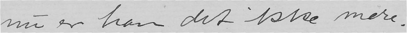nu er han det ikke mere.
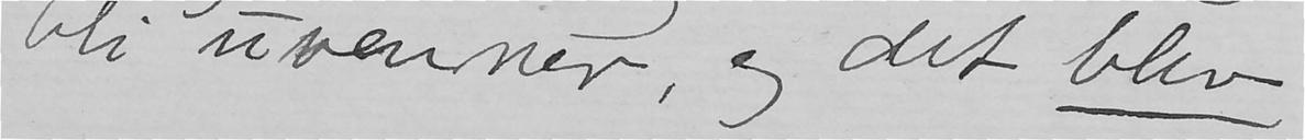bli uvenner, og det blev
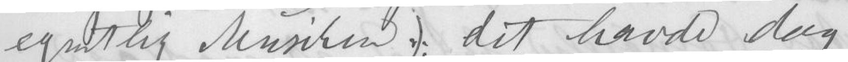egentlig Musiken); det havde dog
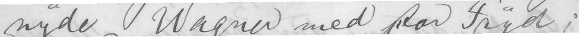nyde Wagner med stor Fryd;
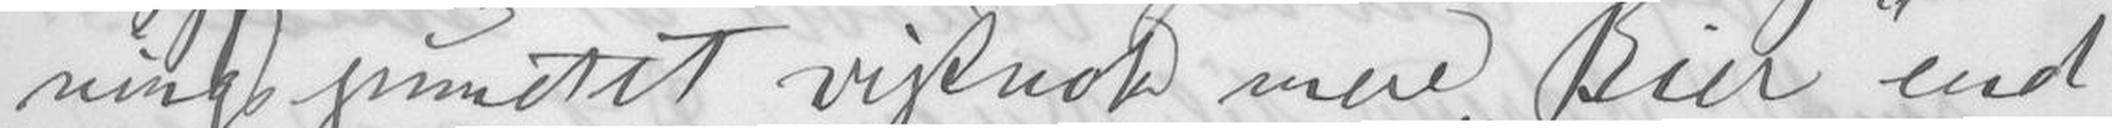ningspunktet vistnok mere Bier end
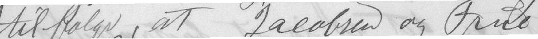tilfølge, at Jacobsen og Frue
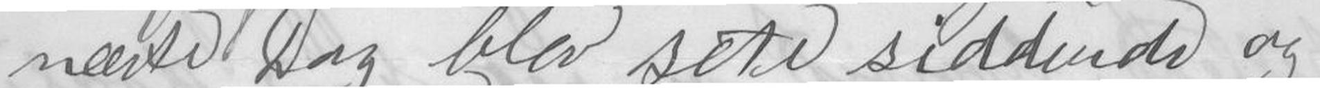næste Dag blev sete siddende og
